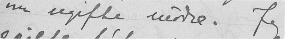om ugifte mødre. Jeg
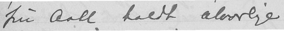fru Aall holdt alvorlige
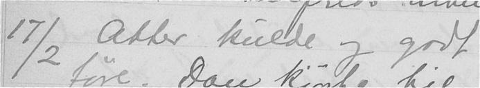17/2 Atter kulde og godt
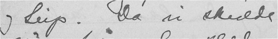og Seip. Da vi skulde
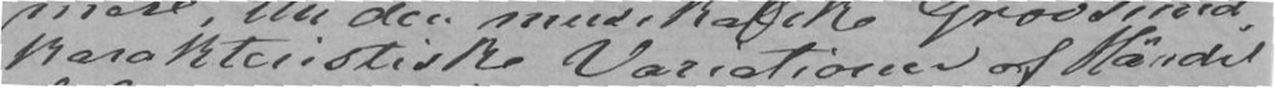karakteristiske Variationer af Händel
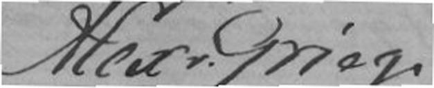Alexr Grieg
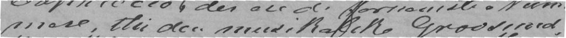mere, thi den musikalske Grovsmed,
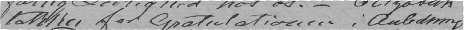takker for Gratulationen i Anledning
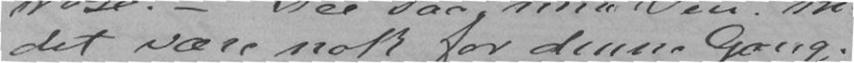det være nok for denne Gang.
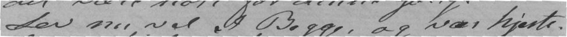Lev nu vel I Begge, og vær hjerte-
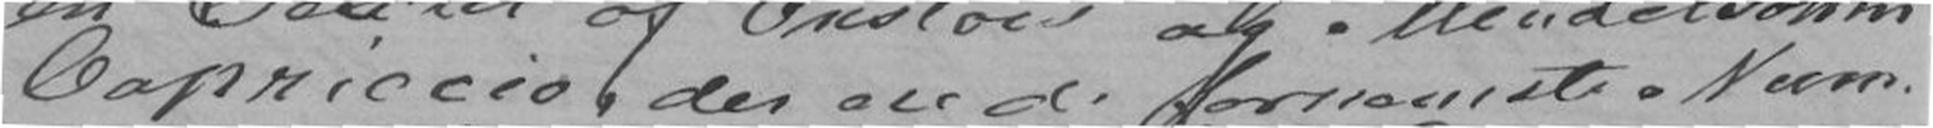Capriccio, der ere de fornæmste Num-
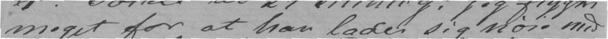meget for at han lader sig nøie med
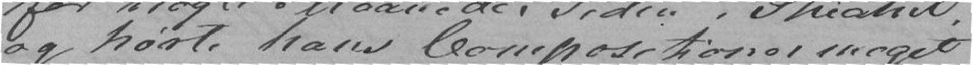og hørte hans Compositioner meget
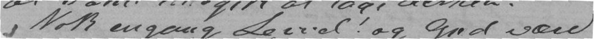Nok engang Levvel! og Gud være
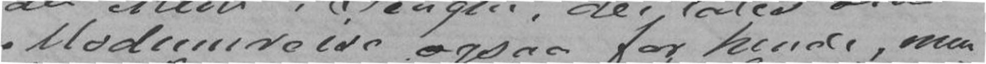Modunreise ogsaa for hende, men
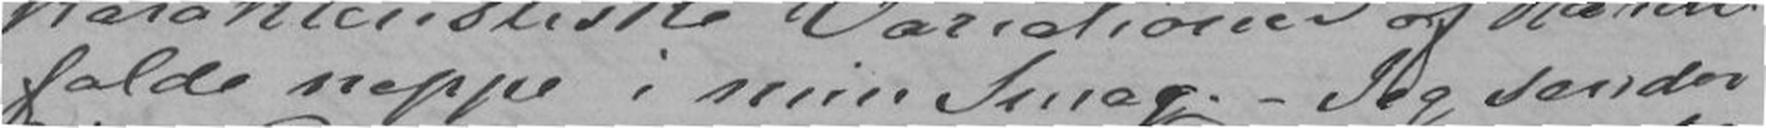falde neppe i min Smag. - Jeg sender
